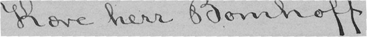Kære herr Bomhoff!
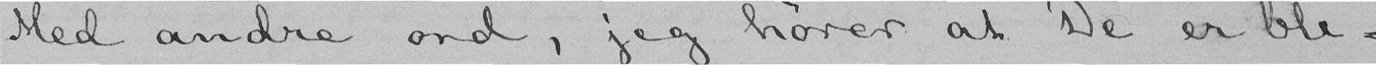Med andre ord, jeg hører at De er ble-
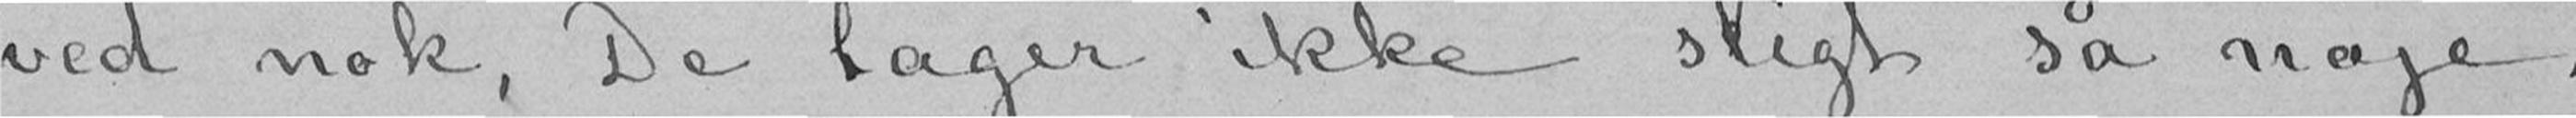ved nok, De tager ikke sligt så nøje.
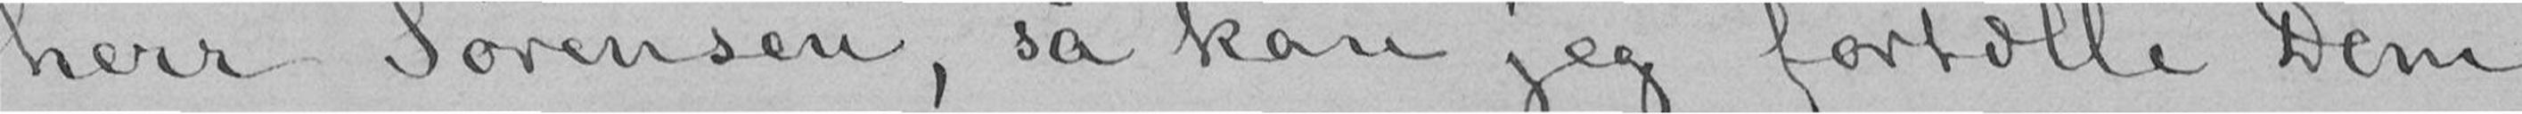herr Sørensen, så kan jeg fortælle Dem
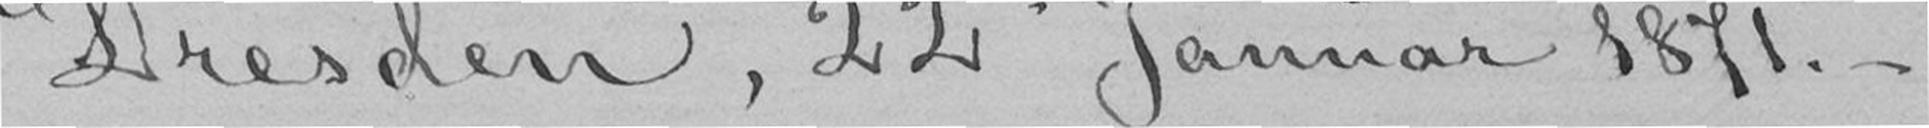Dresden, 22de Januar 1871.-
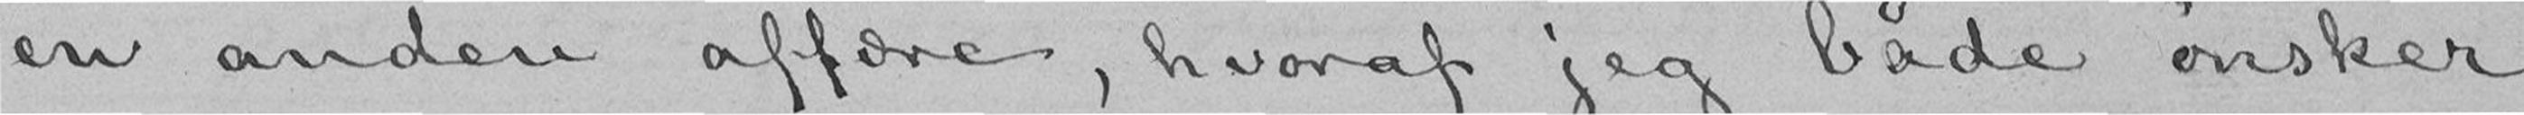en anden affære, hvoraf jeg både ønsker
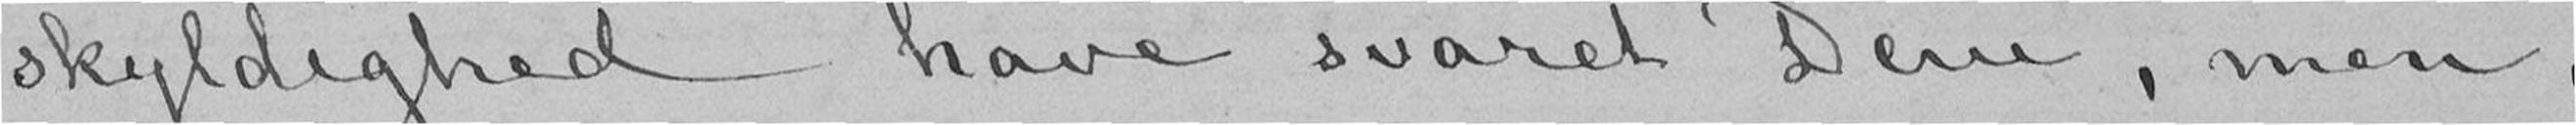skyldighed have svaret Dem, men,
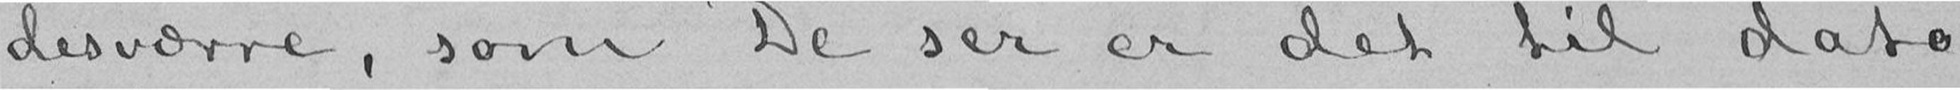desværre, som De ser er det til dato
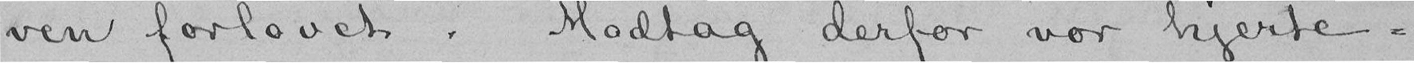ven forlovet. Modtag derfor vor hjerte-
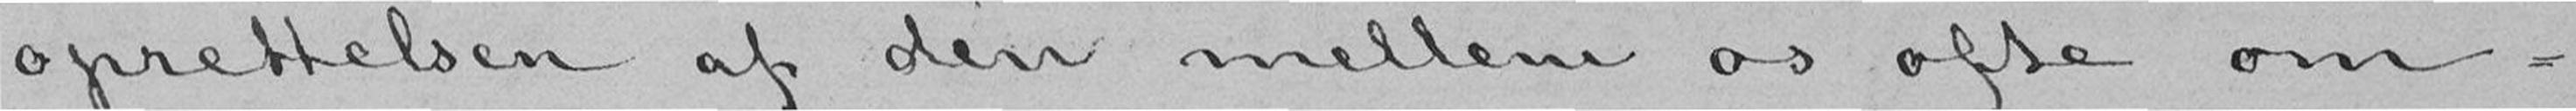oprettelsen af den mellem os ofte om-
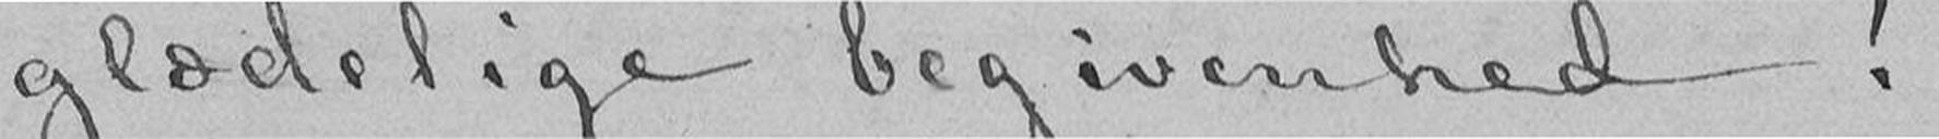glædelige begivenhed!-
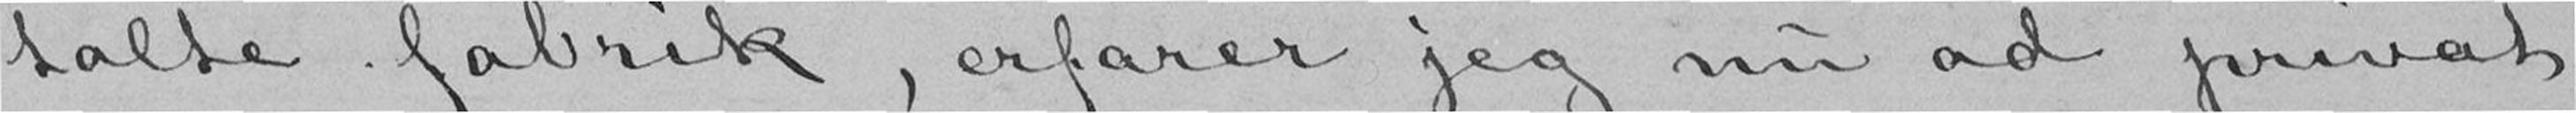talte fabrik, erfarer jeg nu ad privat
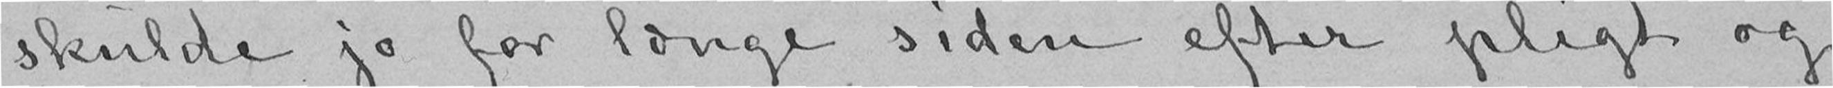skulde jo for længe siden efter pligt og
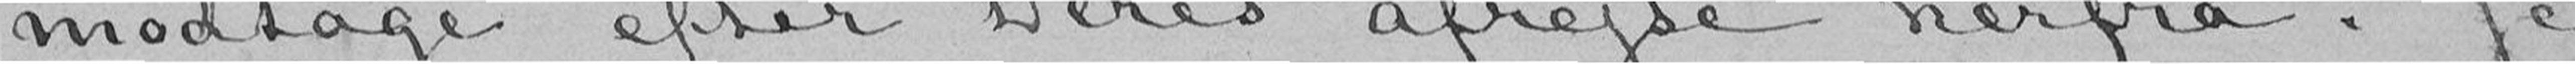modtage efter Deres afrejse herfra. Jeg
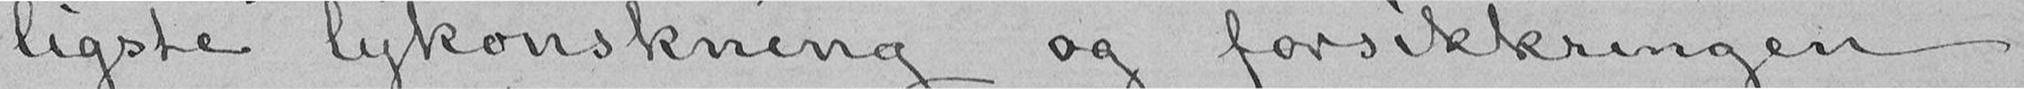ligste lykønskning og forsikkringen
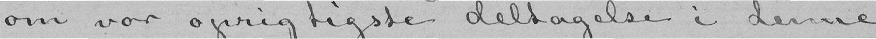om vor oprigtigste deltagelse i denne
